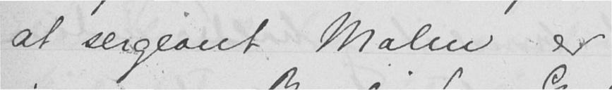at sergeant Malm er
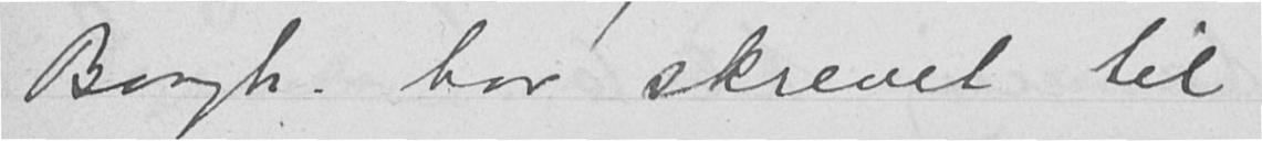Borgh. har skrevet til
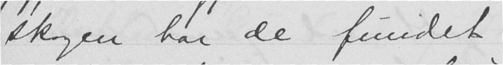skogen har de fundet
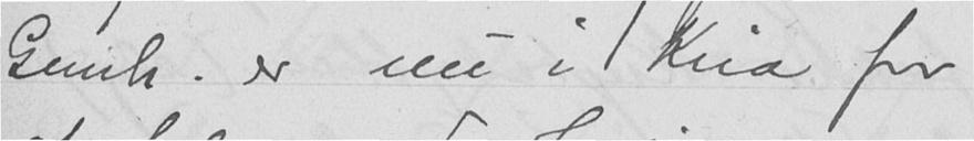Gunh. er nu i Kria for
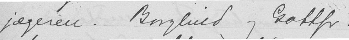jægeren. Borghild og Gottfr.
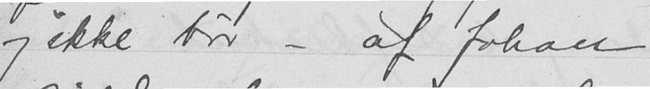og ikke bør - at Johan
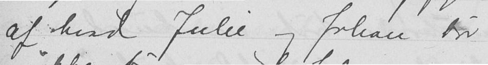af hvad Julie og Johan bør
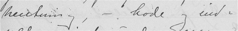hentning, - hode og ind-
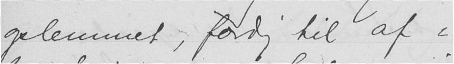oplemmet, ferdig til af-
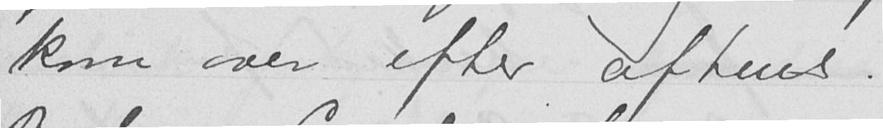kom over efter aftens.
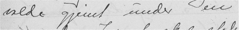volde gjemt under en
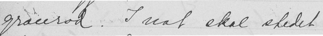granrod. I nat skal stedet
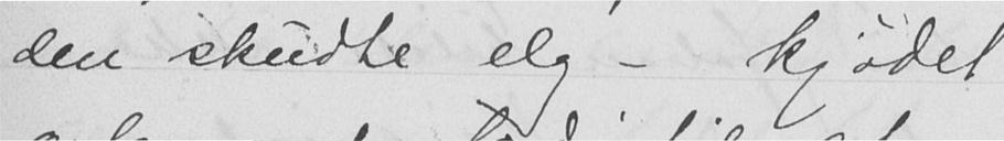den skudte elg - kjødet
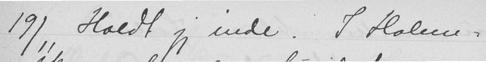19/11 Holdt jeg inde. I Holme-
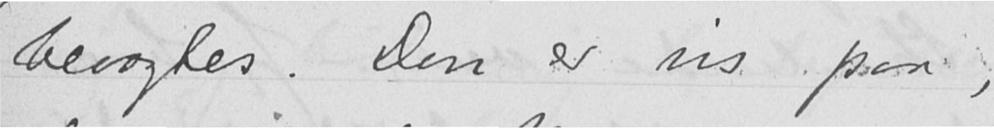bevogtes. Don er vis paa,
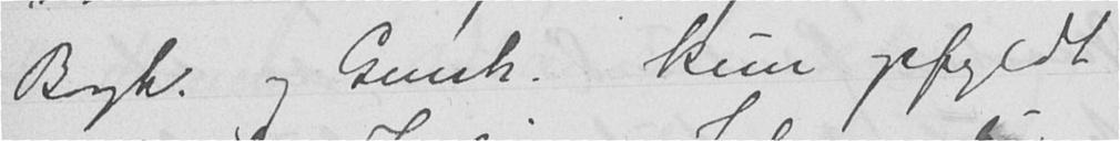Borgh. og Gunh. kun opfyldt
